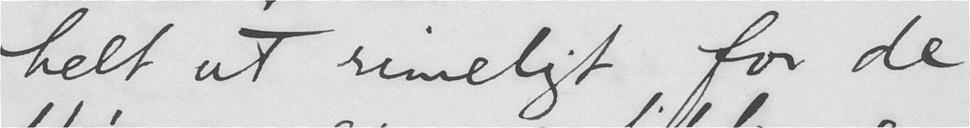helt ut rimelig for de
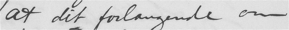at dit forlangende om
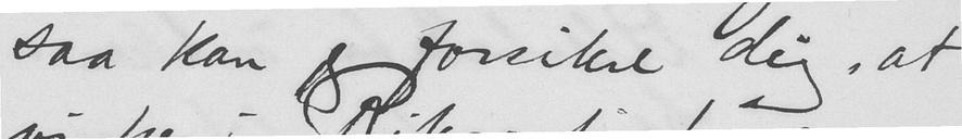da kan jeg forsikre dig, at
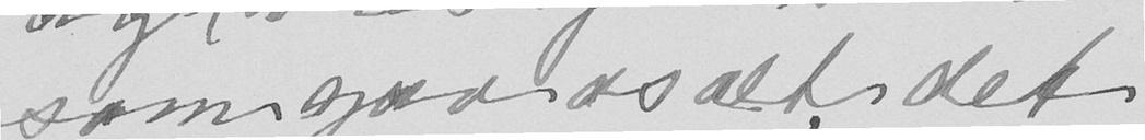som gav os alt det
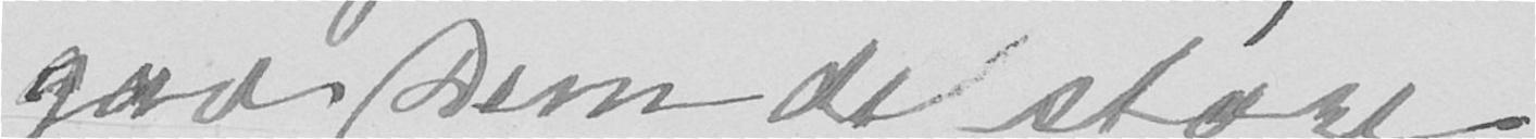gav Dem de store
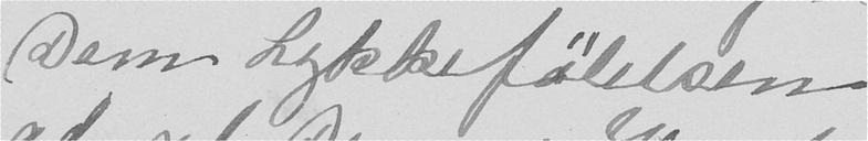Dem Lykkefølelsen
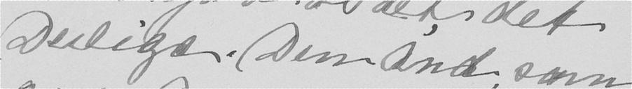Deilige. Den Ånd, som
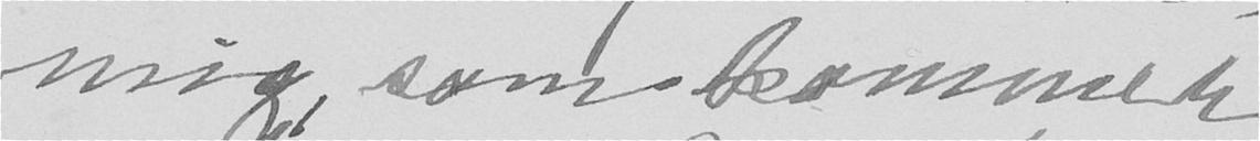mig, som kommer
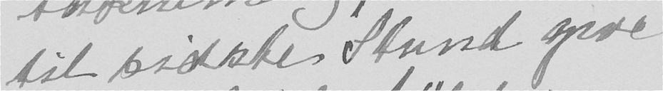til sidste Stund give
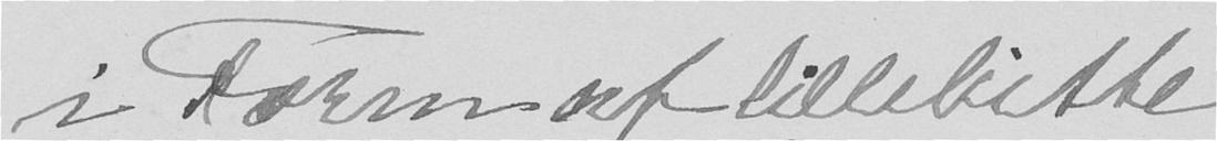i Form af lillebitte
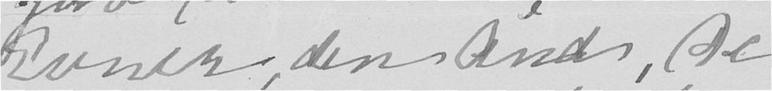Evner, den Ånd, De
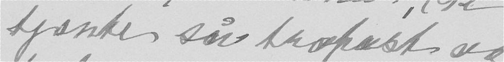tjænte så trofast og
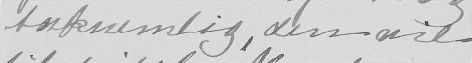taknemlig, den vil
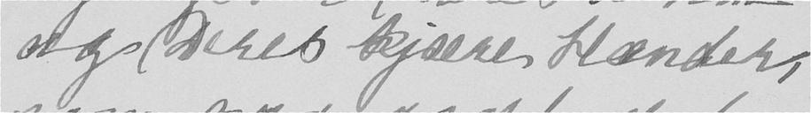og deres kjære Hænder,
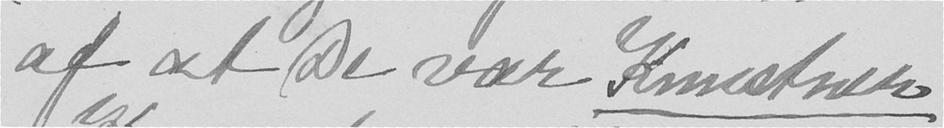af at De var Kunstner.
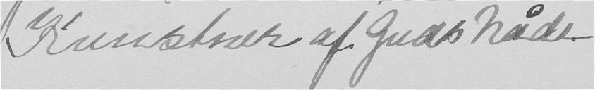Kunstner af Guds Nåde.
